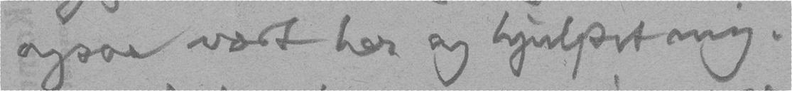ogsaa vært her og hjulpet mig.
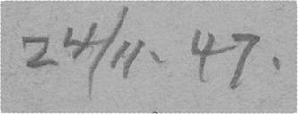24/11. 47.
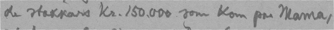de stakkars Kr. 150.000 som kom paa Mama,
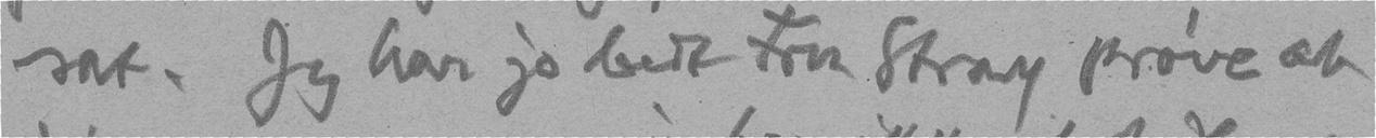sat. Jeg har jo bedt Fru Stray prøve at
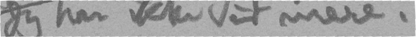Jeg har ikke Tid mere.
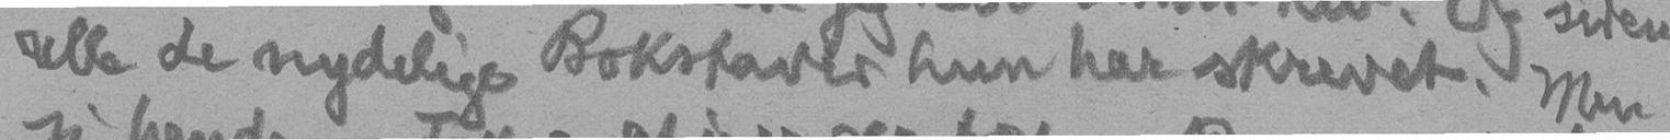alle de nydelige Bokstaver hun har skrevet. Men
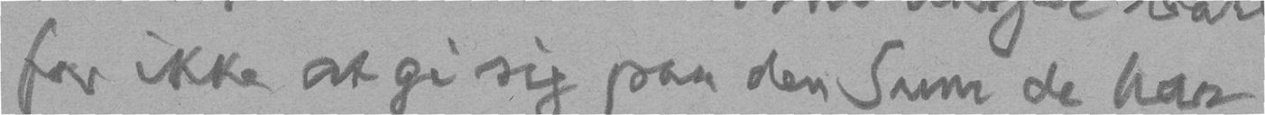for ikke at gi sig paa den Sum de har
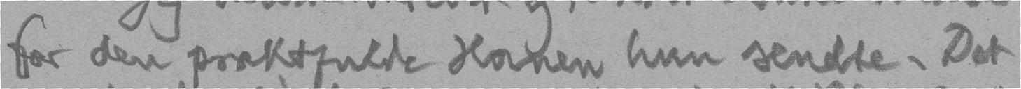for den praktfulde Hanen hun sendte. Det
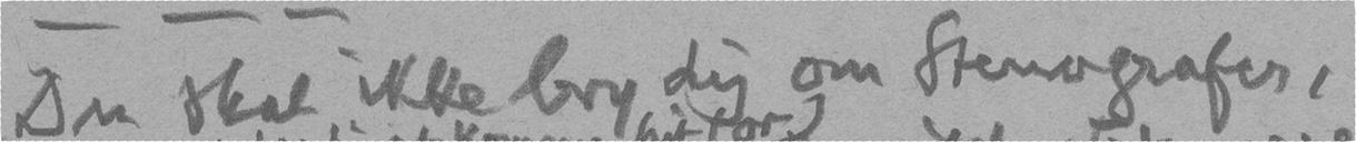Du skal ikke bry dig om Stenografer,
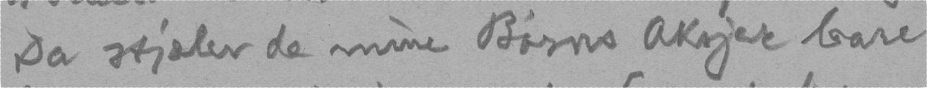Da stjæler de mine Børns Aksjer bare
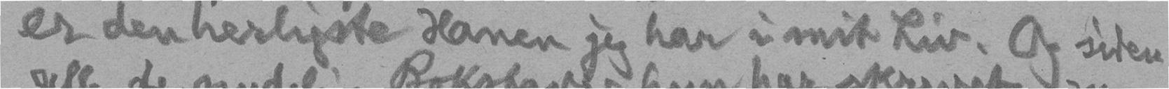er den herligste Hanen jeg har i mit Liv. Og siden
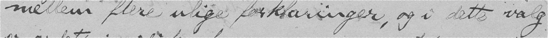mellem flere ulige forklaringer, og i dette valg
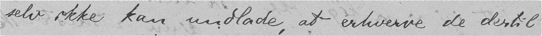selv ikke kan undlade, at erhverve de dertil
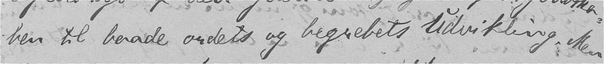ben til baade ordets og begrebets Udvikling. Men
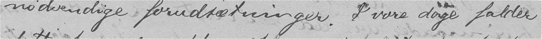nødvendige forudsætninger. I vore dage falder
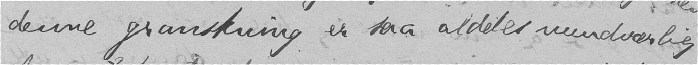denne granskning er saa aldeles uundværlig
